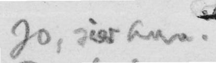Jo, sier hun.
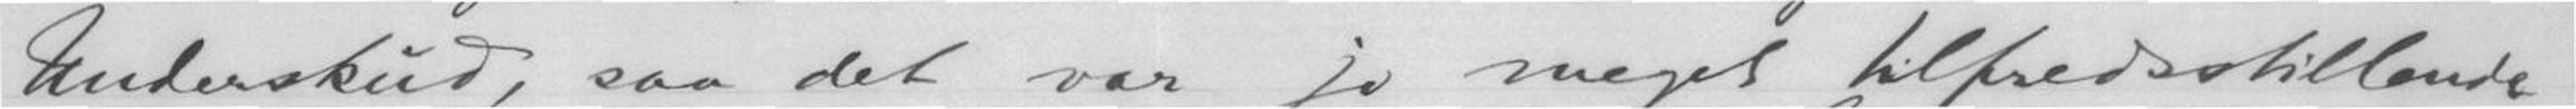Underskud, saa det var jo meget tilfredsstillende.
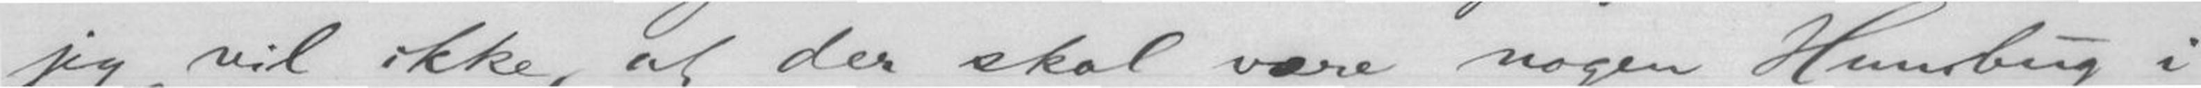jeg vil ikke, at der skal være nogen Humbug i
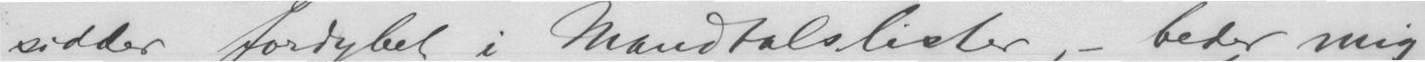sidder fordybet i Mandtalslister, - beder mig
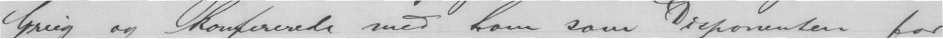Grieg og konfererede med ham som Disponenten for
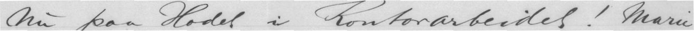Nu paa Hodet i Kontorarbeidet! Marie
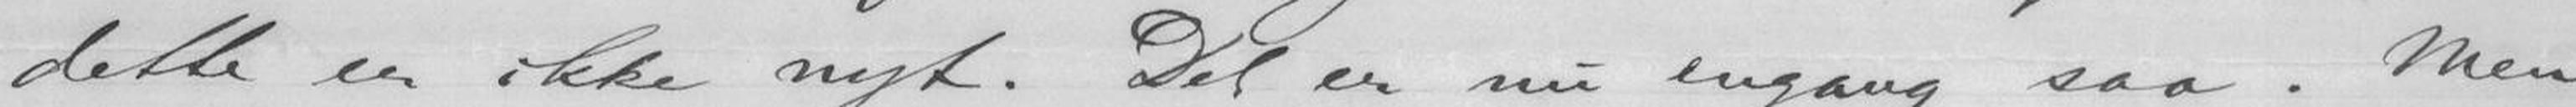dette er ikke nyt. Det er nu engang saa. Men
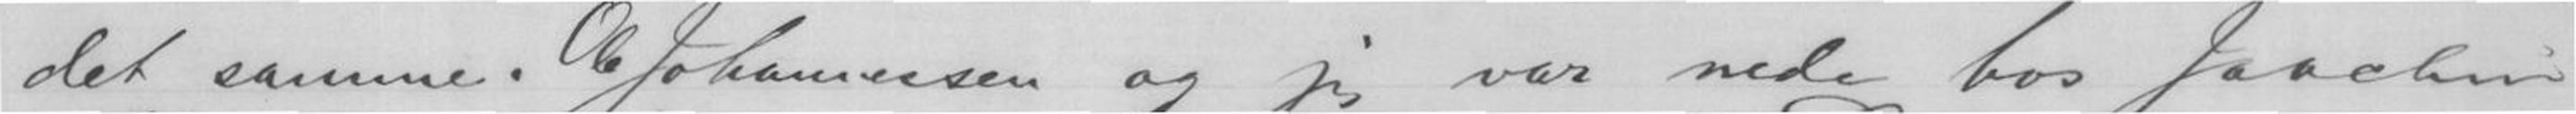det samme. Ole Johannesen og jeg var nede hos Joachim
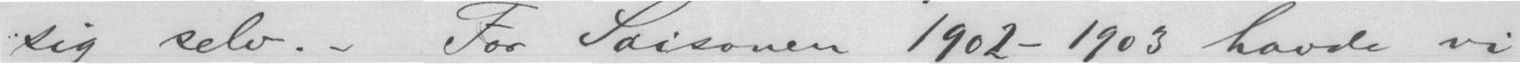sig selv. - For Saisonen 1902-1903 havde vi
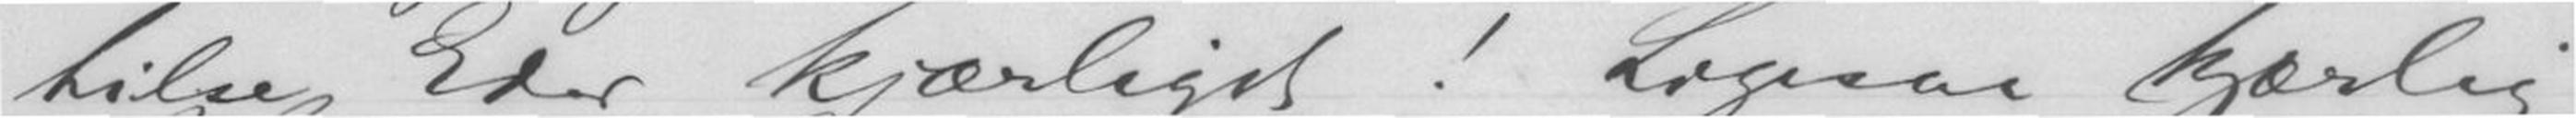hilse Eder kjærligst! Ligesaa kjærlig
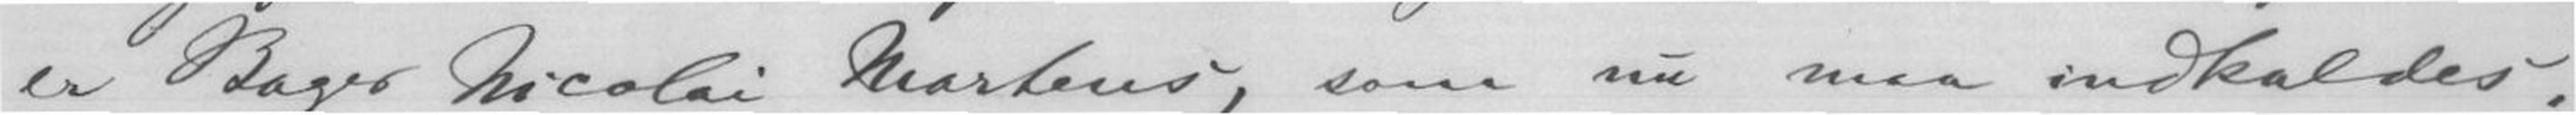er Bager Nicolai Martens, som nu maa Indkaldes.
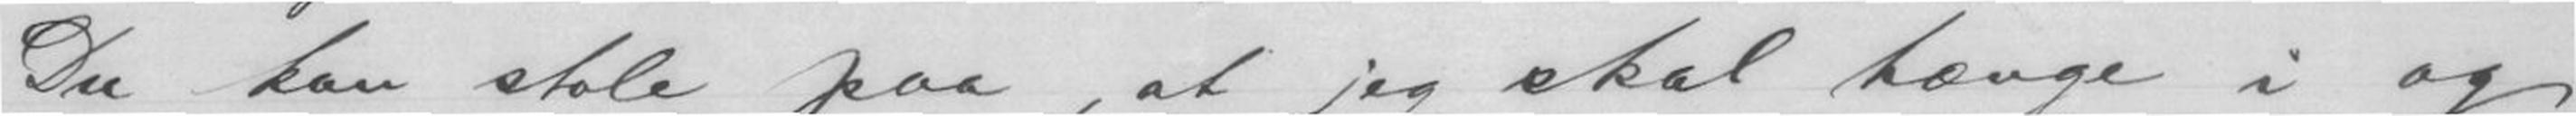Du kan stole paa, at jeg skal hænge i og
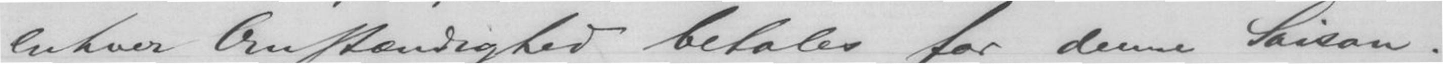enhver Omstændighed betales for denne Saison.
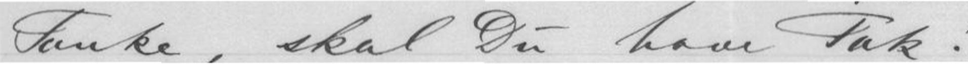Tanke, skal Du have Tak!
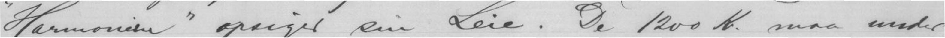"Harmonien" opsiger sin Leie. De 1200 Kr. maa under
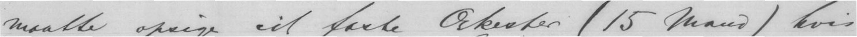maatte opsige sit faste Orkester (15 Mand) hvis
